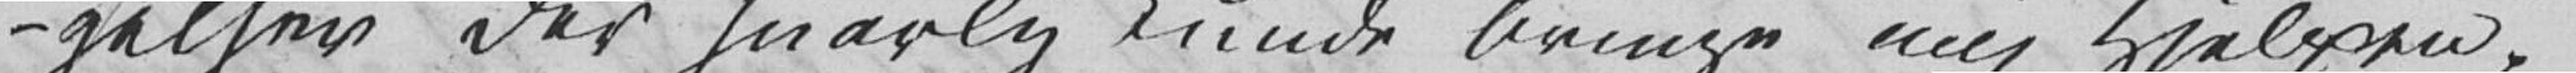gelser der snarlig kunde bringe mig Hjelpen.
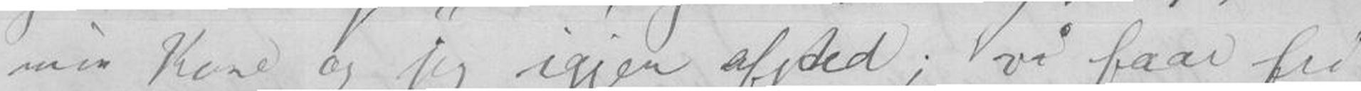min Kone og jeg igjen afsted; vi faar fri
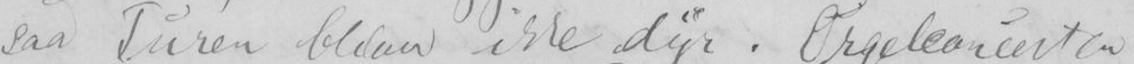saa Turen bliver ikke dyr. Orgelconcerten
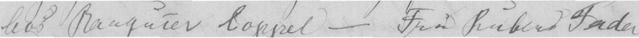hos Banquier Coppel - Fru Rubens Fader,
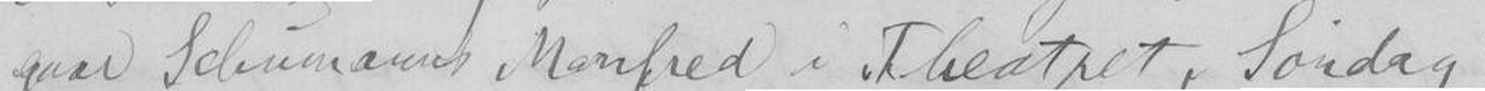gaar Schumanns Manfred i Theatret, Søndag
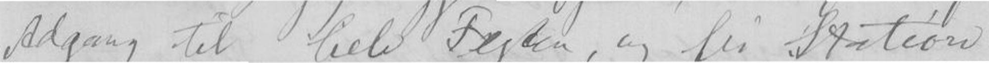Adgang til hele Festen, og fri Station
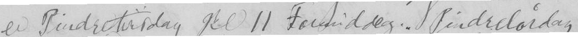er Pindsetirsdag Kl 11 Formiddag. Pindselørdag
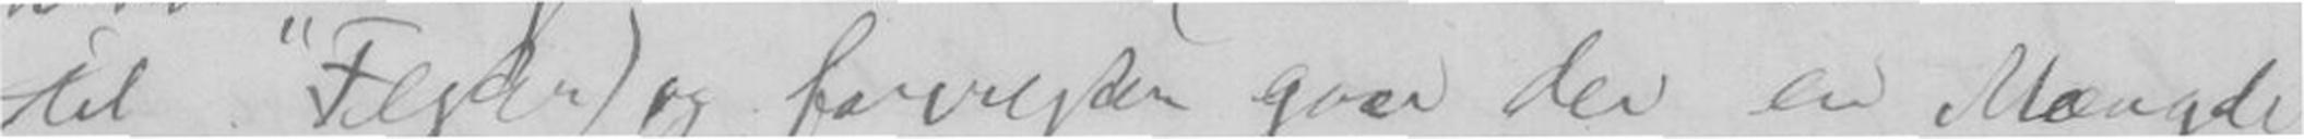til Festen) og forresten gaar der en Mængde
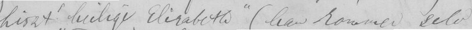Liszt heilige Elisabeth (han kommer selv
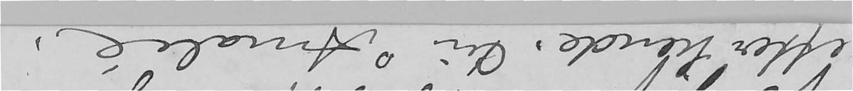efter hende. Din Amalie.
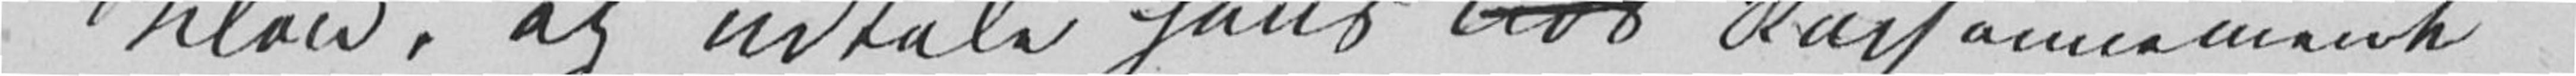Stilen, og udtale hans Tids Raisonnements
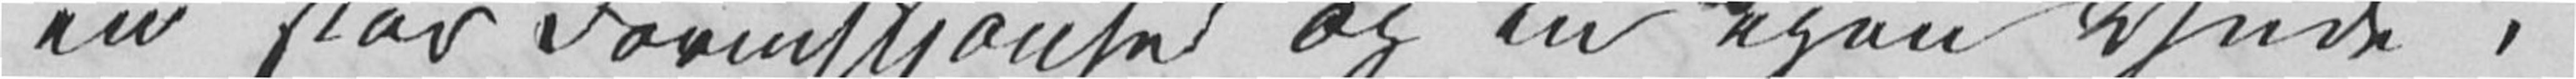en stor Formskjønhed og en egen Ynde i
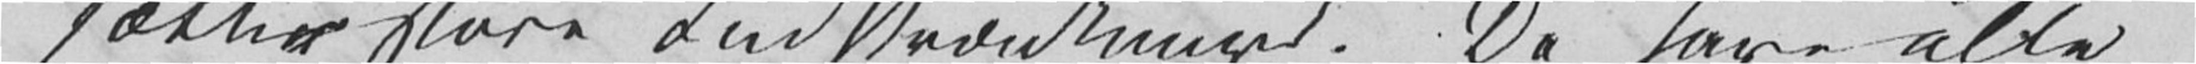sætter store Indskrænkninger. De have alle
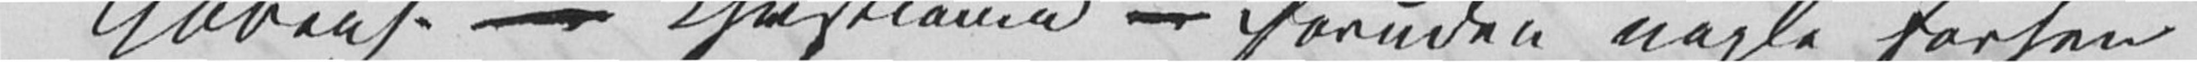Kjøbenh. – Christiania – foruden nogle forhen
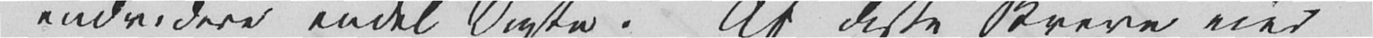endvidere endel Digte. Af disse Breve eier
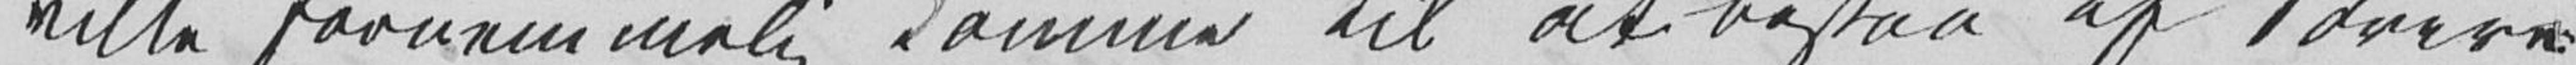ville fornemmelig komme til at bestaa af Breve
